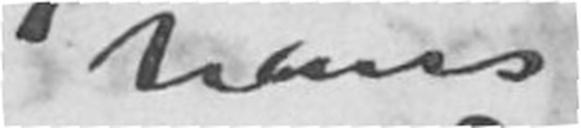hans
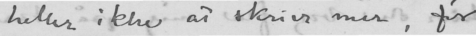heller ikke at skrive mer, før
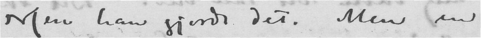Men han gjorde det. Men en
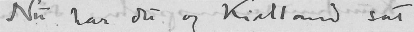Nu har du og Kielland sat
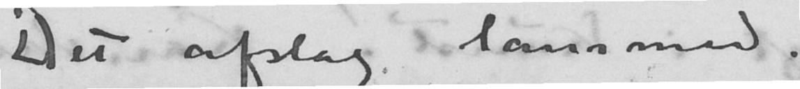Det afslag lammed.
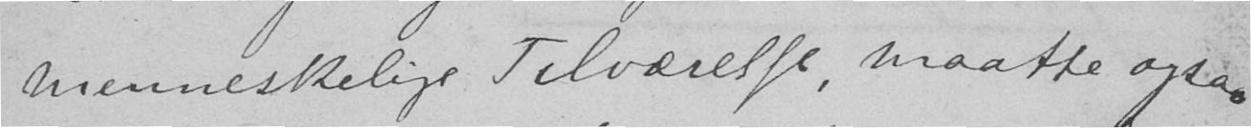menneskelige Tilværelse, maatte ogsaa
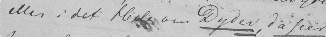eller i det Hele om Dyder, da seer
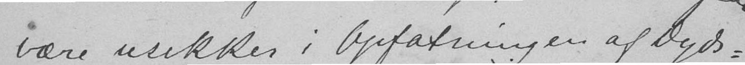være usikker i Opfatningen af Dyds-
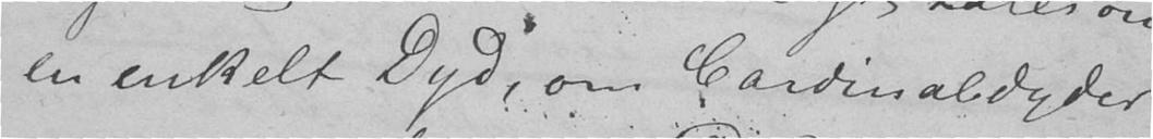en enkelt Dyd, om Cardinaldyder
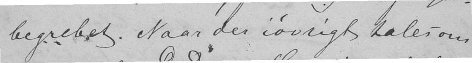begrebet. Naar der iøvrigt tales om
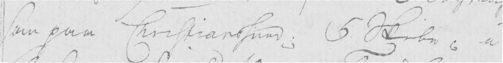som paa Christianssund; 5 Skibe; i
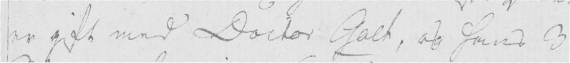er gift med Doctor Galt, og hans 3
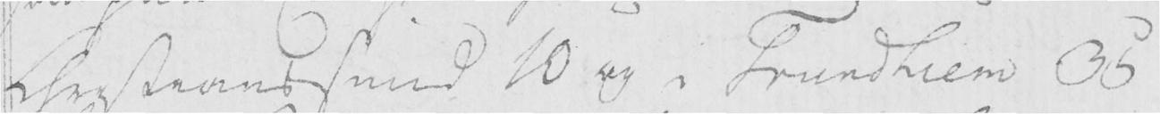Christianssund 10 og i Trundhiem 35
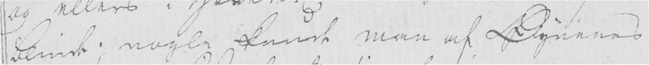blinde; nogle kunde man af Øynenes
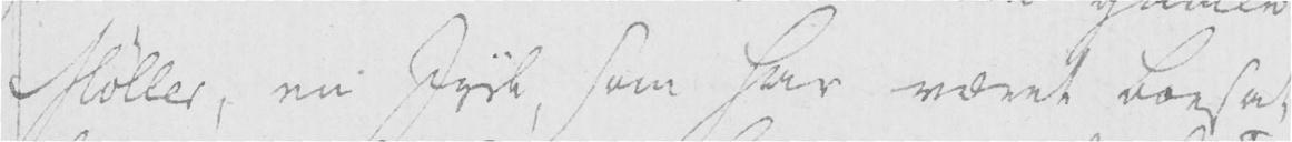Møller, en Jysk, som har været boesat
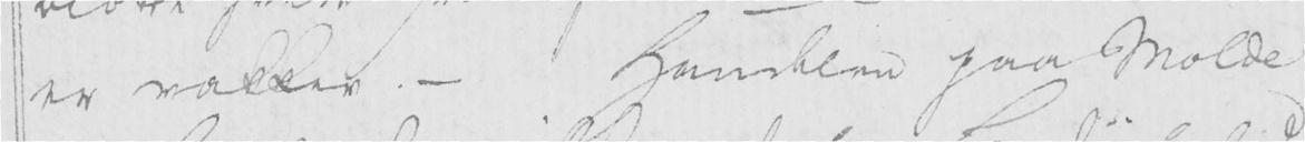er vakker. - Handelen paa Molde
